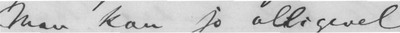Man kan jo alligevel
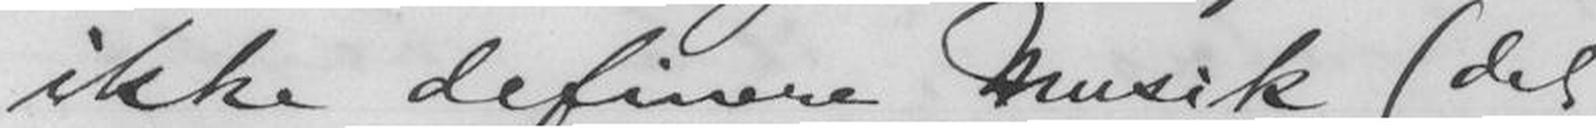ikke definere Musik (det
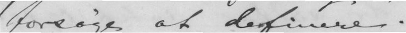forsøge at definere.
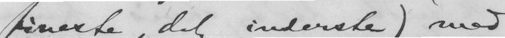fineste, det inderste) med
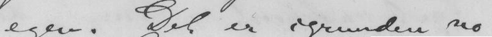egen. Det er igrunden no-
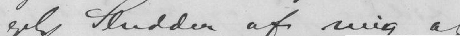get Sludder af mig at
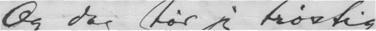Og dog tør jeg trøstig
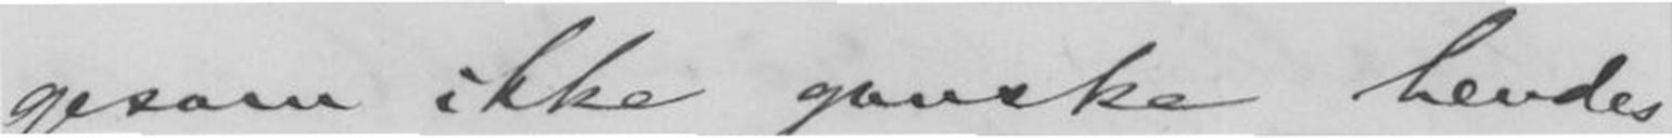gesom ikke ganske hendes
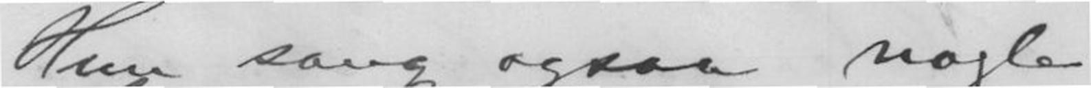Hun sang ogsaa nogle
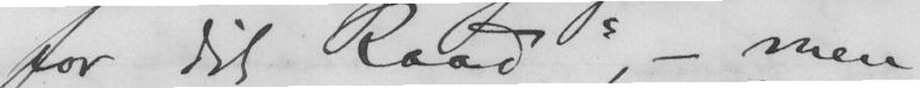for dit Raad", - men
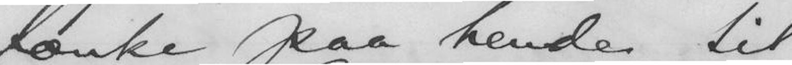tænke paa hende til
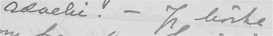rævehi. - Jeg hørte
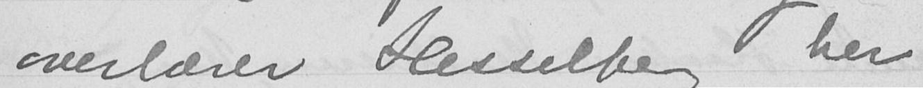overlærer Hesselberg her
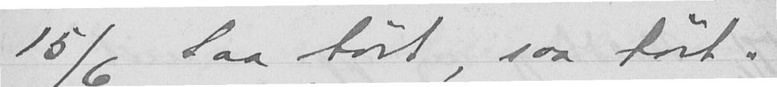15/6 Saa tørt, saa tørt.
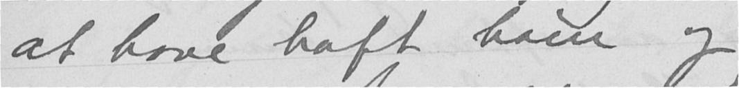at have haft ham og
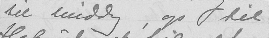til middag, op til
